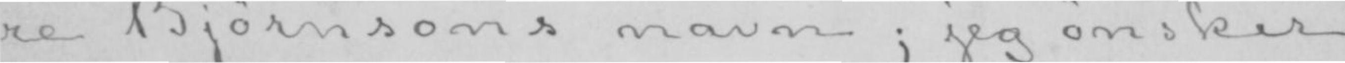re Bjørnsons navn; jeg ønsker
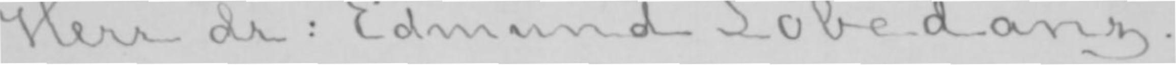Herr dr: Edmund Lobedanz.
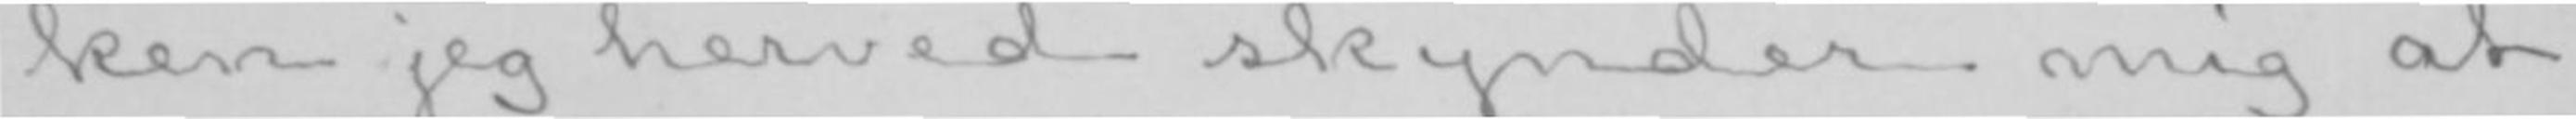ken jeg herved skynder mig at
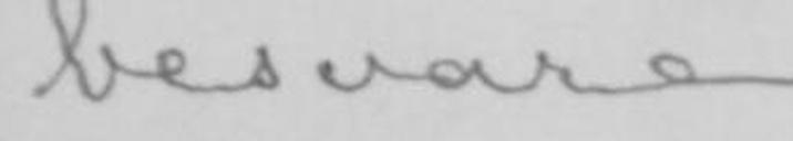besvare.
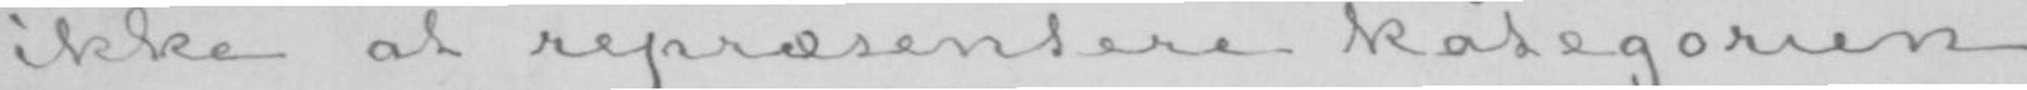ikke at repræsentere kategorien
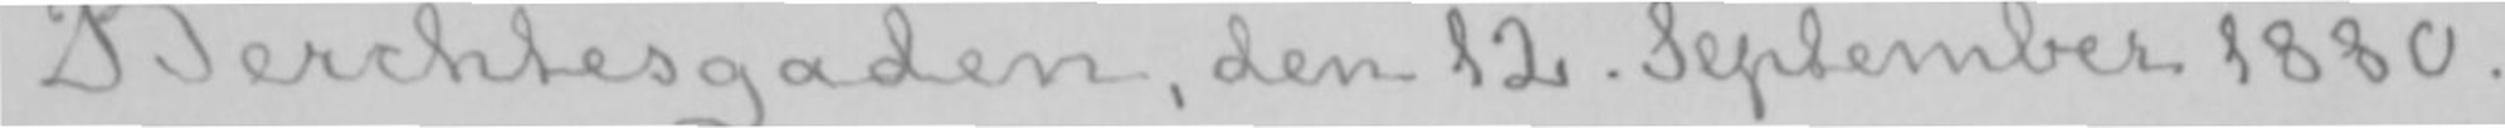Berchtesgaden, den 12. September 1880.
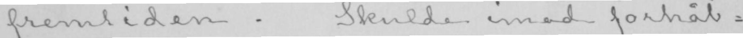fremtiden. Skulde imod forhåb-
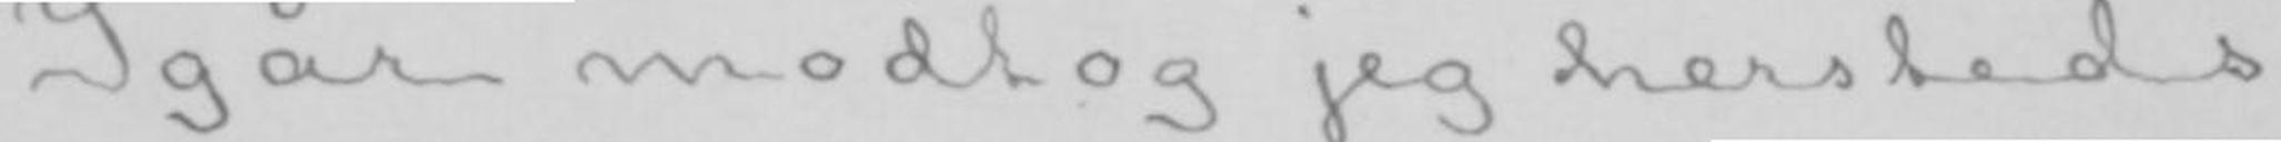Igår modtog jeg hersteds
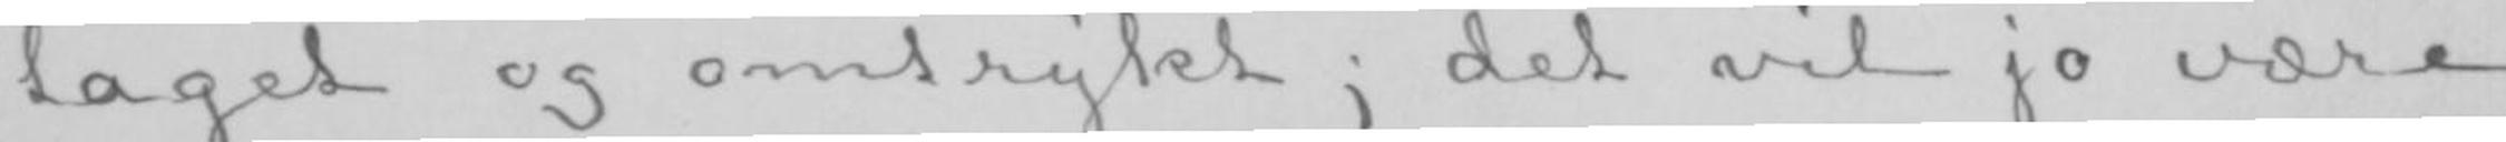taget og omtrykt; det vil jo være
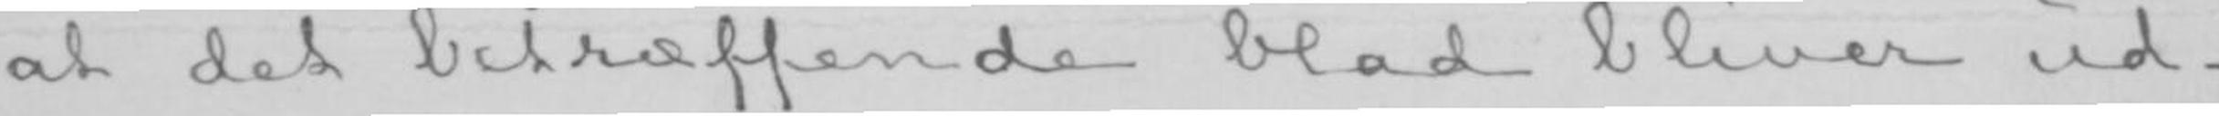at det betræffende blad bliver ud-
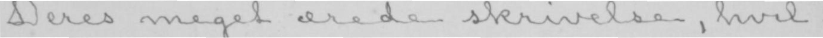Deres meget ærede skrivelse, hvil-
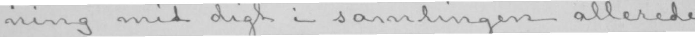ning mit digt i samlingen allerede
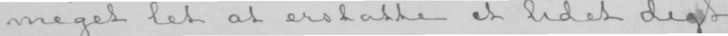meget let at erstatte et lidet digt
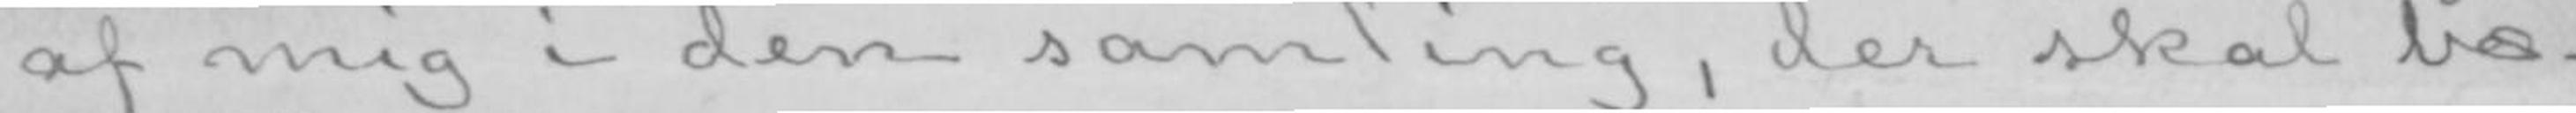af mig i den samling, der skal bæ-
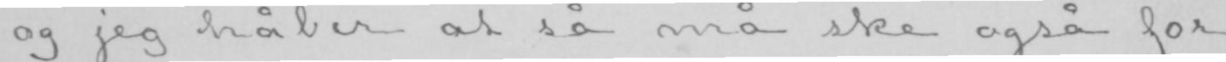og jeg håber at så må ske også for
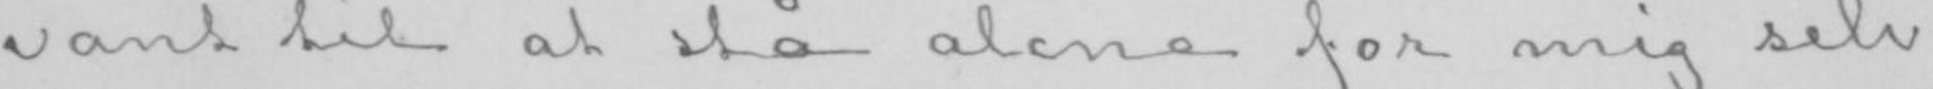vant til at stå alene for mig selv,
